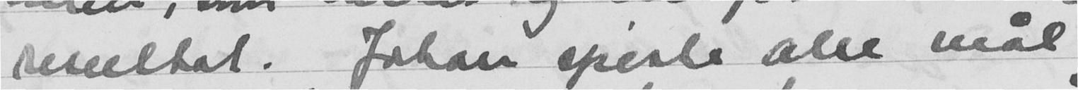resultat. Johan spiste alle mål
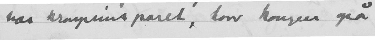hos kronprinsparet, hvor kongen også
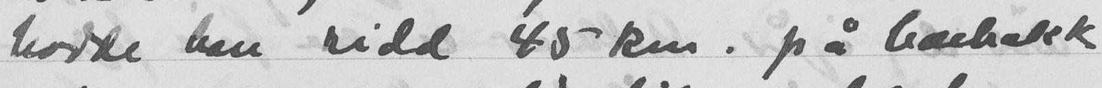bodde han ridd 45 km. på barbakk
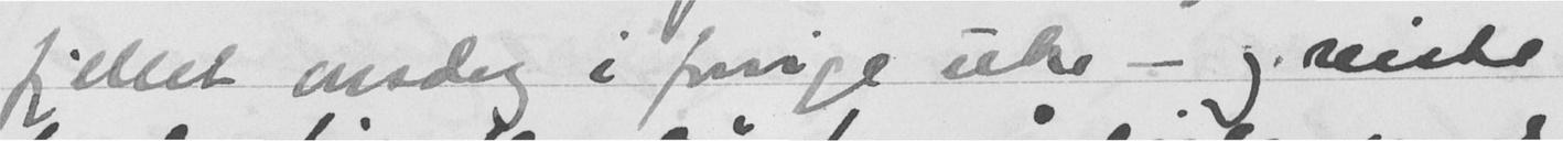fjellet mandag i forrige uke - og reiste
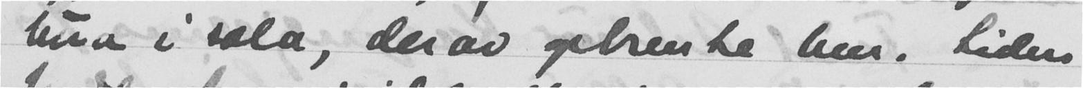bua i sola, der av ophente hus. Siden
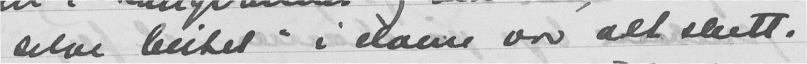selve "beitet" i elven var alt slutt.
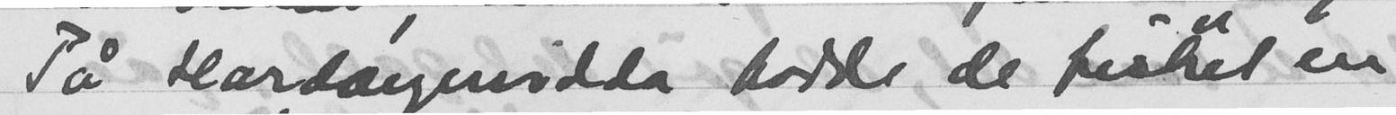På Hardangervidda hadde de fisket en
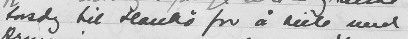torsdag til Hankø for å seile med
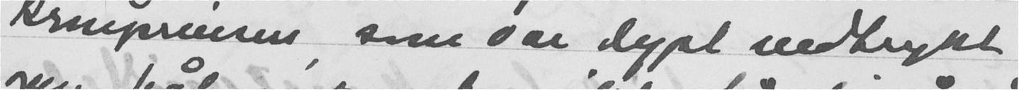kronprinsen, som var dypt nedtrykt
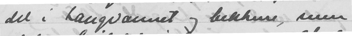del i Langvannet og bikkere, men
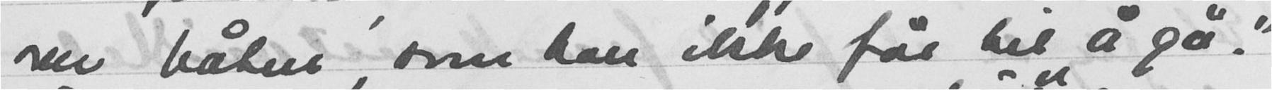over båten, som han ikke får til å gå!
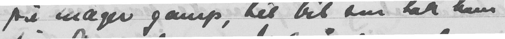på mager gamp, til han tok ham
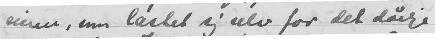reisen, som lastet sig selv for det dårlige
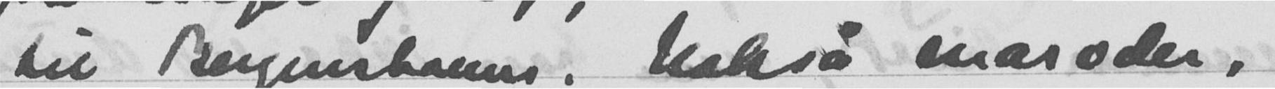til Bergenshavn. Nokså maroder,
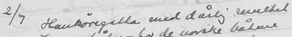2/7 Hankøregatta med dårlig resultat
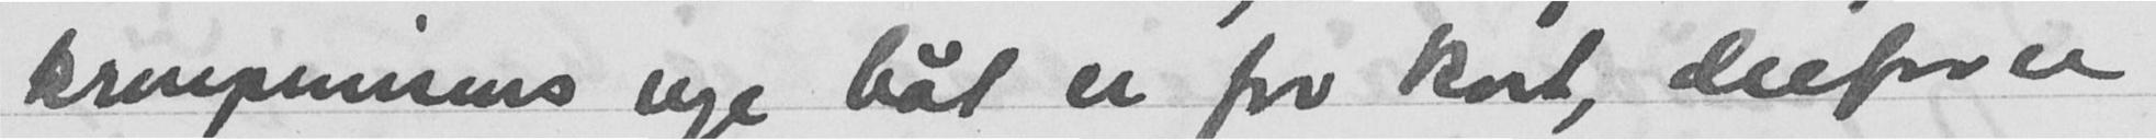kronprinses nye båt er for kort, derfor er
